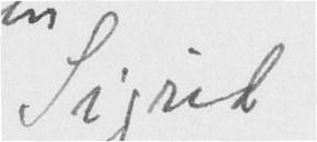Sigrid.
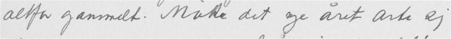altfor gammelt. Måtte det nye året arte sig
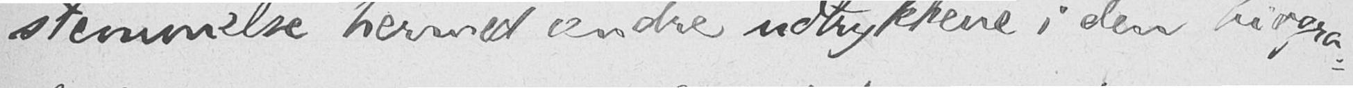stemmelse hermed ændre udtrykkene i den biogra-
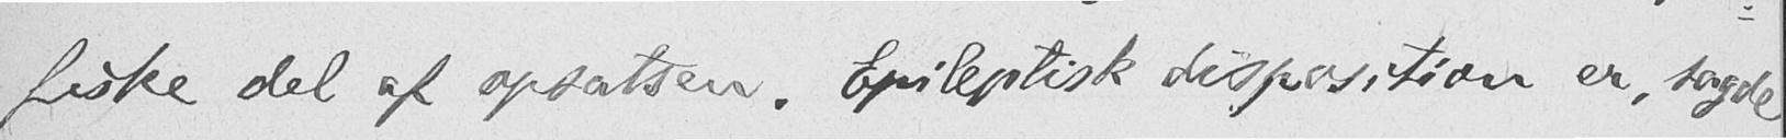fiske del af opsatsen. Epileptisk disposition er, sagde
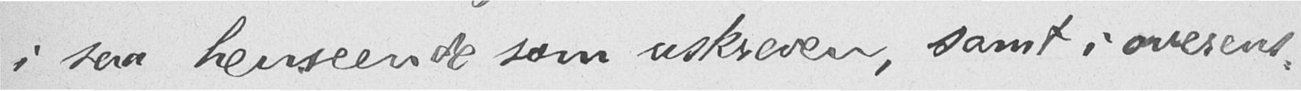i saa henseende som uskreven, samt i overens-
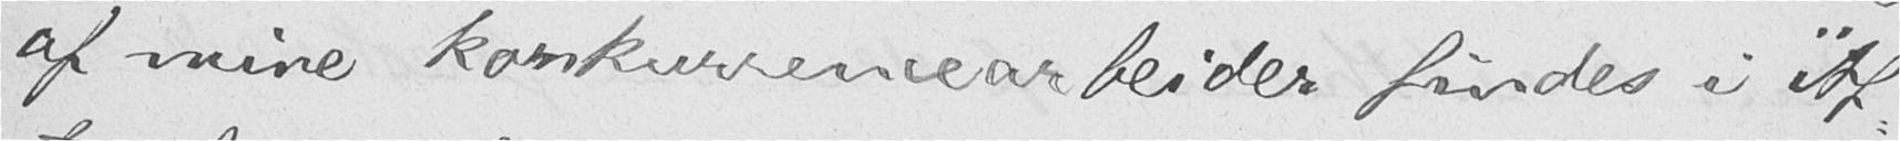af mine konkurrencearbeider findes i "Af-
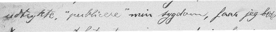udtrykte, "publicere" min sygdom, faar jeg bede
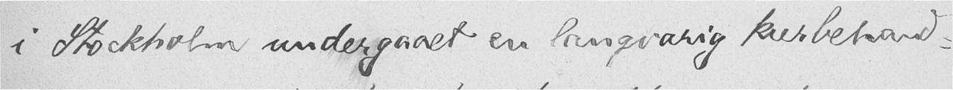i Stockholm undergaaet en langvarig kurbehand-
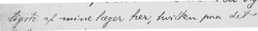tigste af mine læger her, hvilken paa det
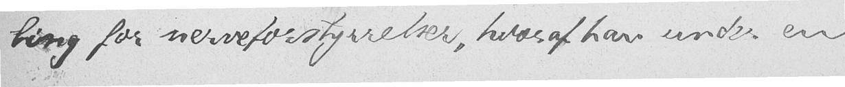ling for nerveforstyrrelser, hvoraf han under en
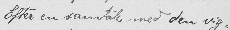Efter en samtale med den vig-
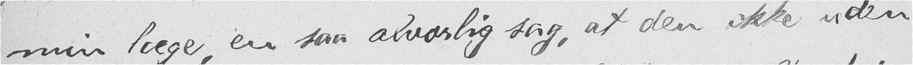min læge, en saa alvorlig sag, at den ikke uden
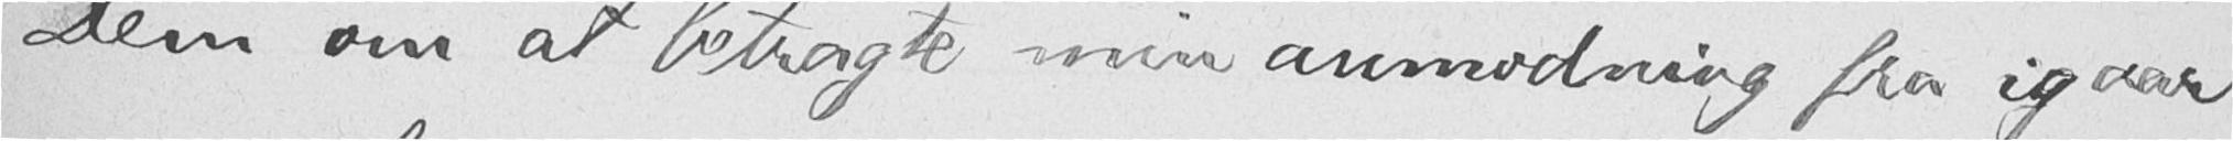Dem om at betragte min anmodning fra igaar
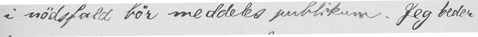i nødsfald bør meddeles publikum. Jeg beder
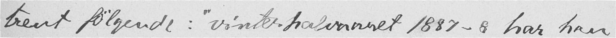trent følgende: "vinterhalvaaret 1887-8 har han
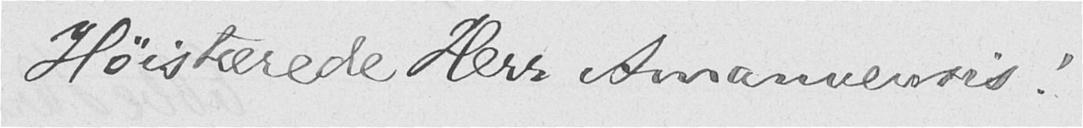Høistærede Herr Amanuensis!
